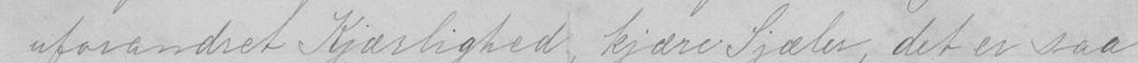uforandret Kjærlighed, kjære Sjæber, det er saa
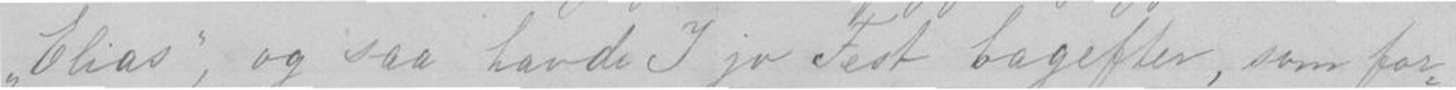"Elias", og saa havde I jo Fest bagefter, som for-
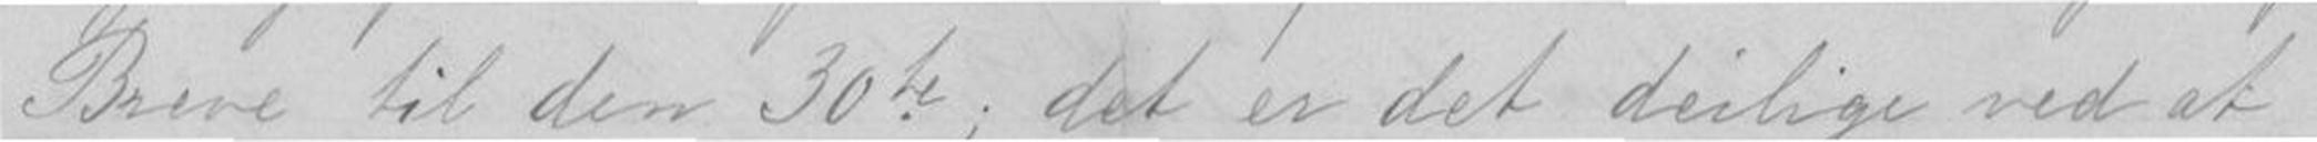Breve til den 30te, det er det deilige ved at
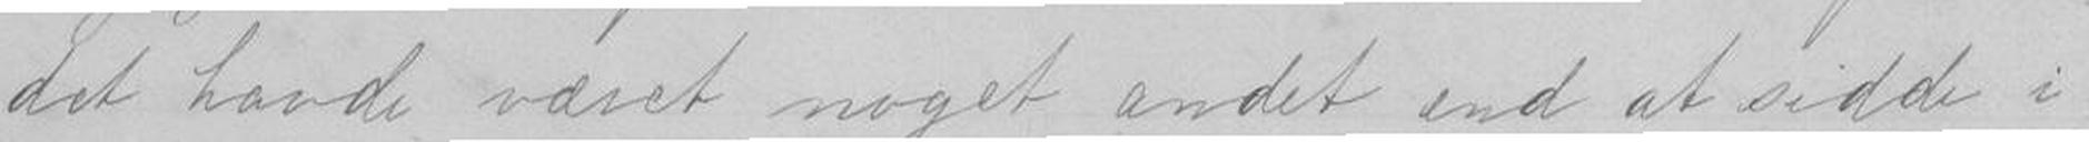det havde været moget andet end at sidde i
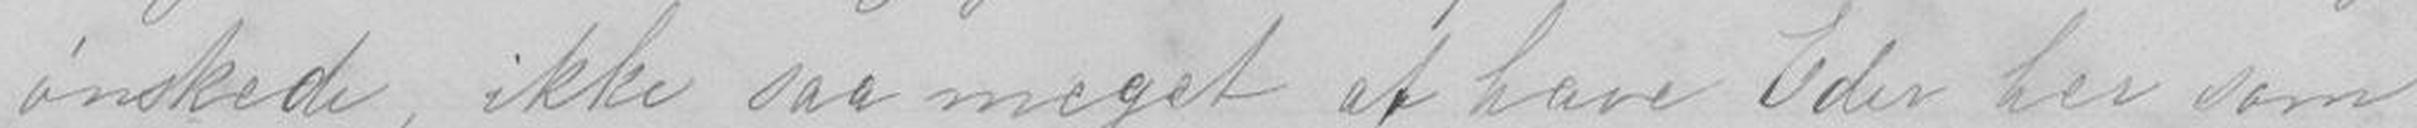ønskede, ikke saa meget at have Eder her som
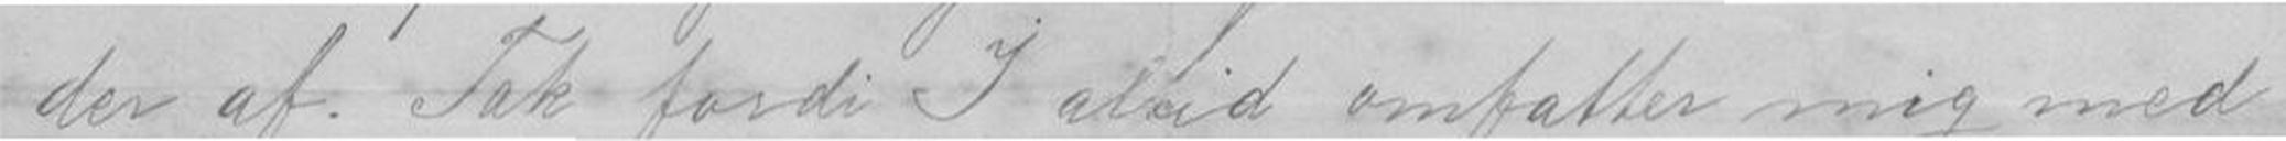der af Tak fordi I alrid omfatter mig med
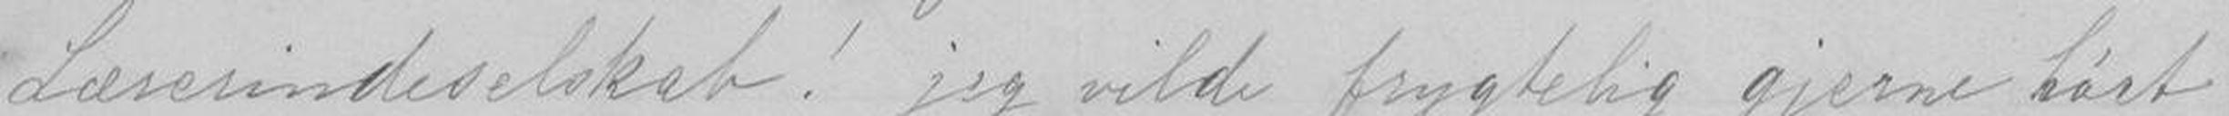Lærerindeselskab! jeg vilde frygtelig gjerne hørt
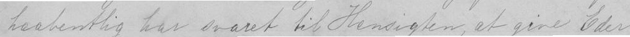haabentlig har svaret til hensigten, at give Eder
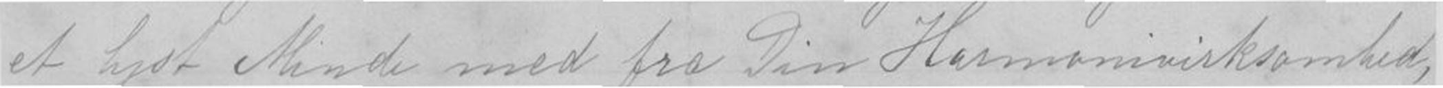et Lyst Minde med fra Din Harmoninisksomled,
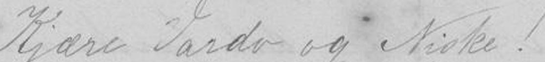Kjære Vardo og Niske!
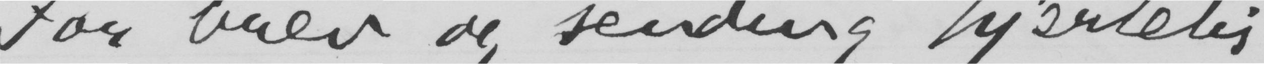For brev og sending hjertelig
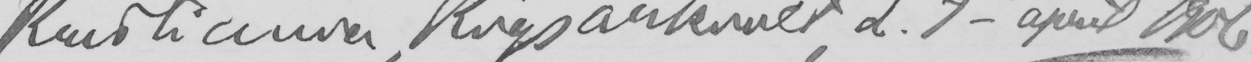Kristiania, Rigsarkivet, d. 7de april 1906.
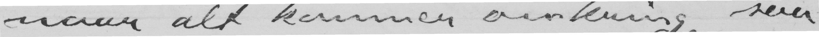naar alt kommer […?], saa
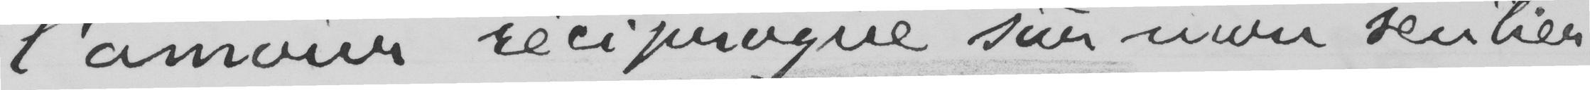l’amour réciproque sur mon sentier.
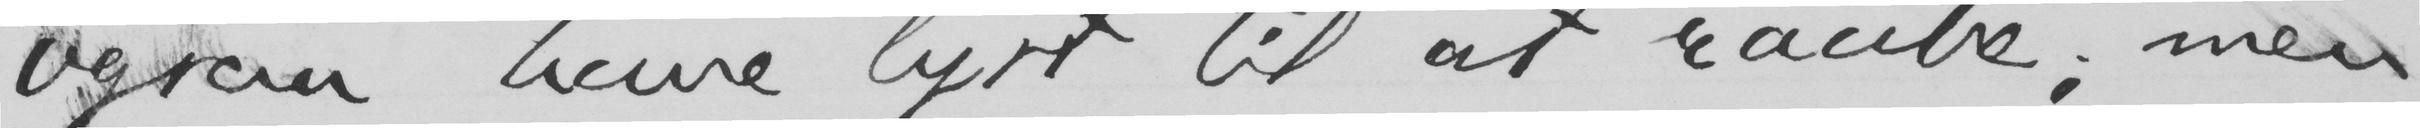ogsaa have lyst til at raabe, men
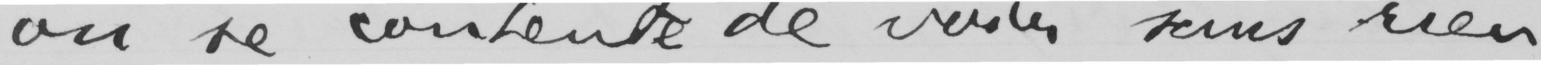on se contente de voir sans rien
